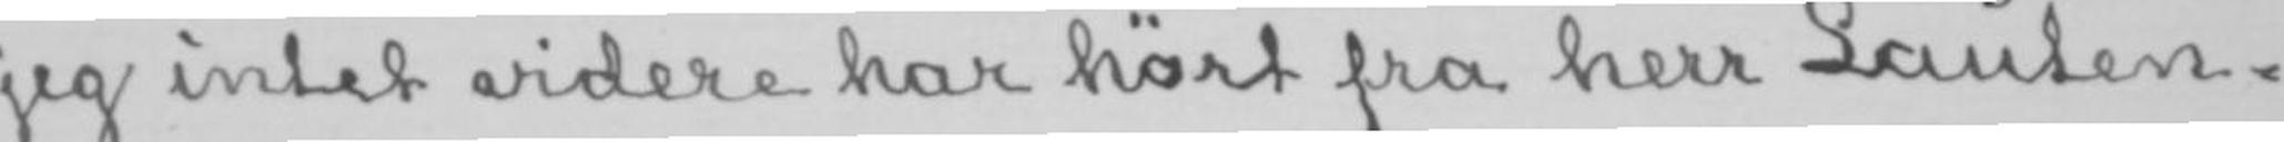jeg intet videre har hørt fra herr Lauten-
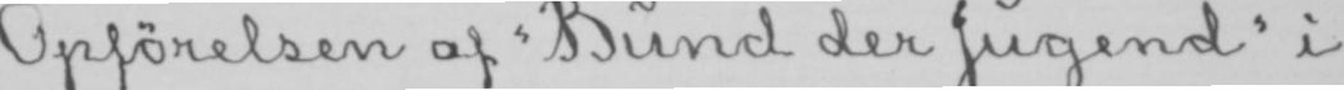Opførelsen af «Bund der Jugend» i
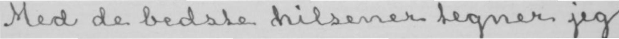Med de bedste hilsener tegner jeg
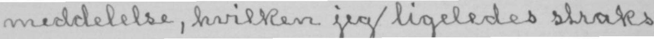meddelelse, hvilken jeg ligeledes straks
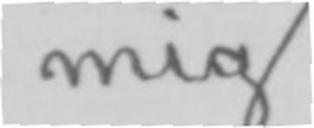mig
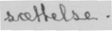sættelse.
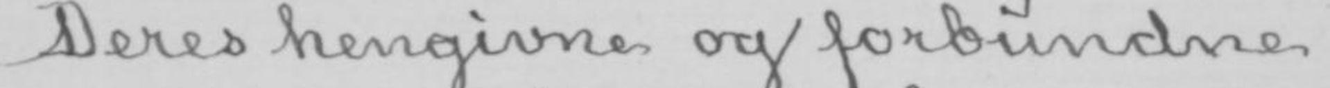Deres hengivne og forbundne
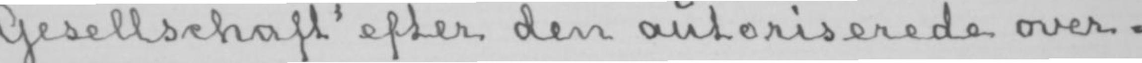Gesellschaft» efter den autoriserede over-
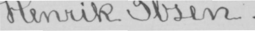Henrik Ibsen.
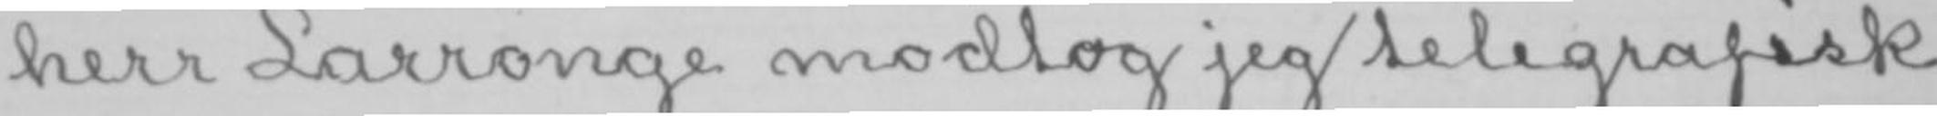herr Larronge modtog jeg telegrafisk
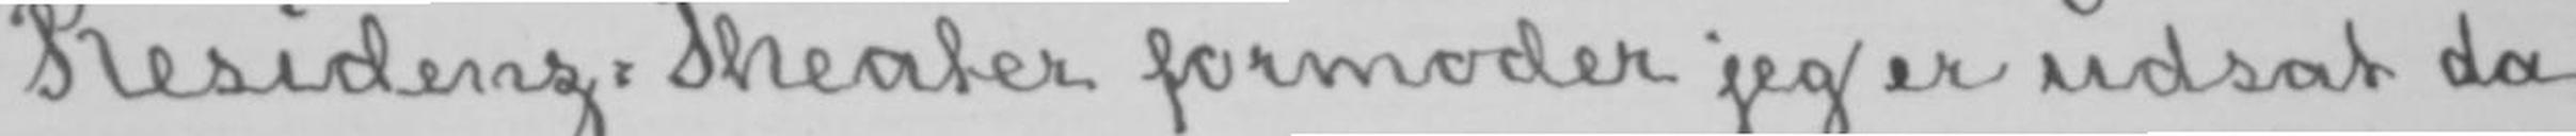Residenz-Theater formoder jeg er udsat da
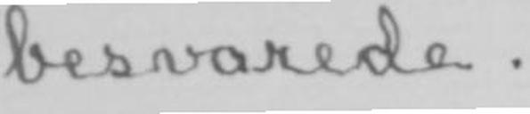besvarede.
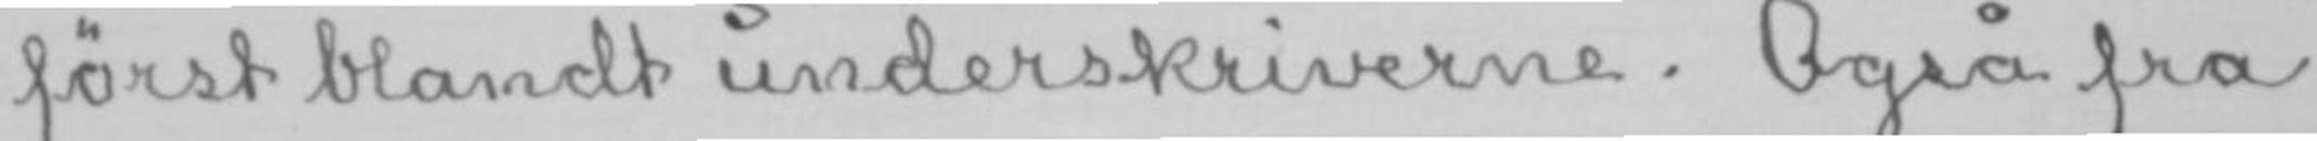først blandt underskriverne. Også fra
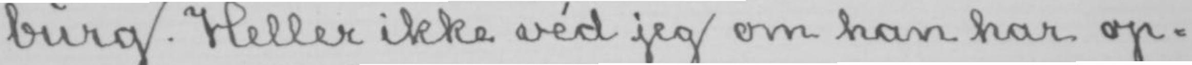burg. Heller ikke véd jeg om han har op-
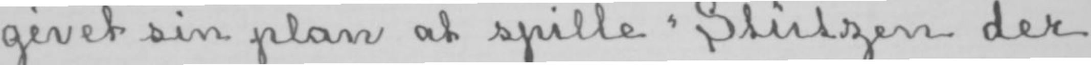givet sin plan at spille «Stützen der
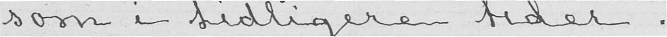som i tidligere tider.
